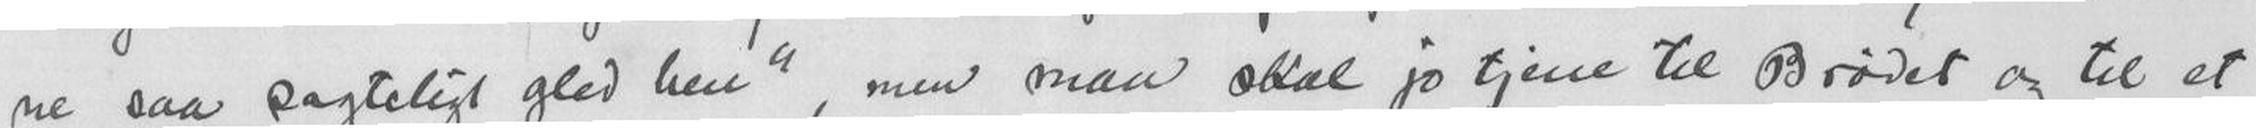ne saa sagteligt gled hen, men man skal jo tjene til Brødet og til et
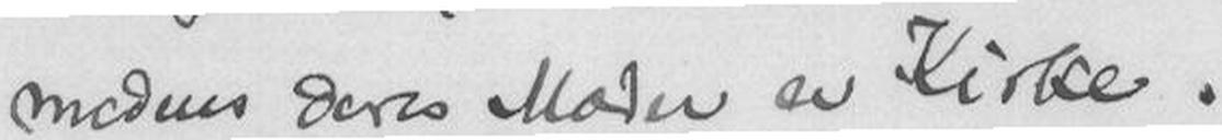medens deres Moder er Kirke.
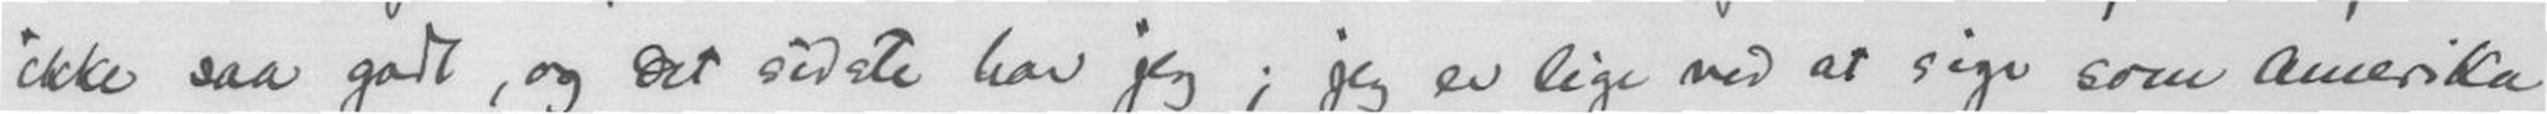ikke saa godt, og det sidste har jeg; jeg er lige ved at sige som Amerika-
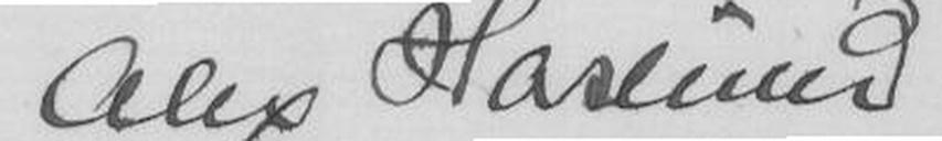Alex Haslund.
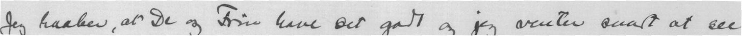Jeg haaber, at De og Frue havde det godt og jeg venter snart at see
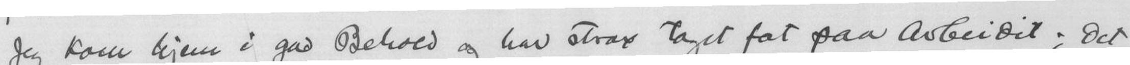Jeg kom hjem i god Behold og har strax taget fat paa Arbeidet; Det
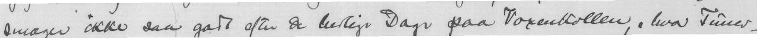smager ikke saa godt efter De herlige Dage paa Voxenkollen, hvor Timer-
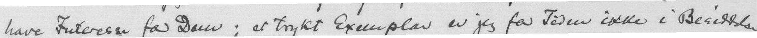have Interesse for Dem; et trykt Exemplar er jeg for Tiden ikke i Besiddelse
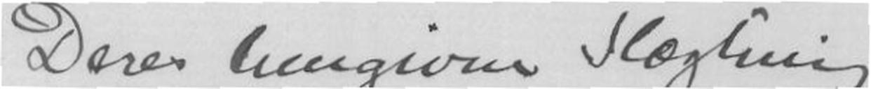Deres hengivne Slægtning
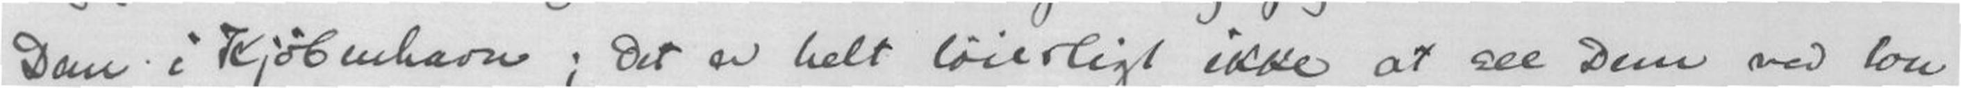Dem i Kjøbenhavn; det er helt løierligt ikke at see Dem ved Con-
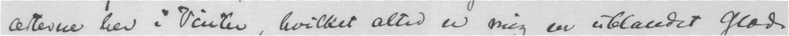certerne her i Vinter, hvilket altid er mig en ublandet glæde
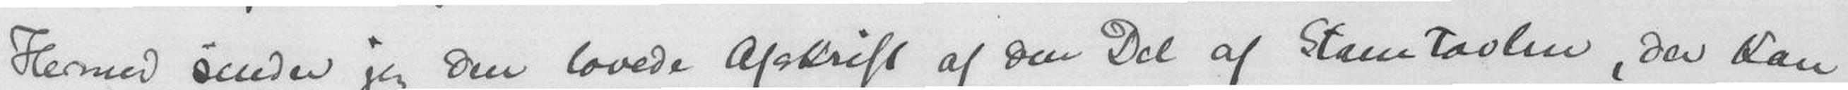Hermed sender jeg Dem lovede Afskrift af den Del af Stam- tavlen, der kan
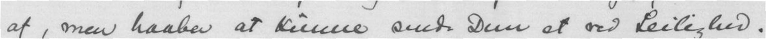af, men haaber at kunne sende Dem et ved Leilighed.
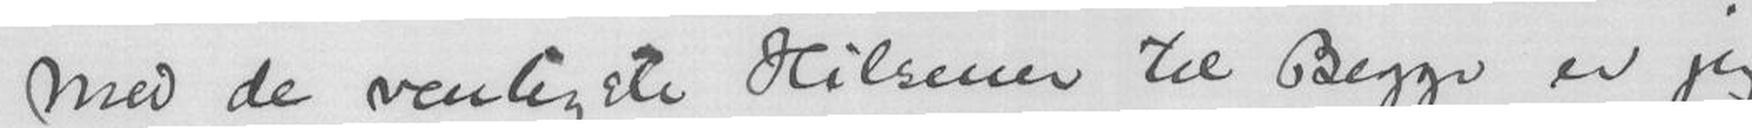Med de venligste Hilsener til Begge er jeg
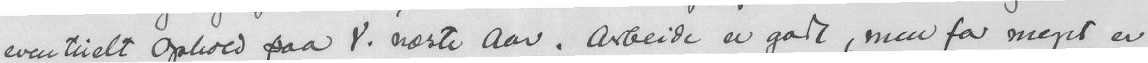eventuelt Ophold paa V. næste Aar. Arbeide er godt, men for meget er
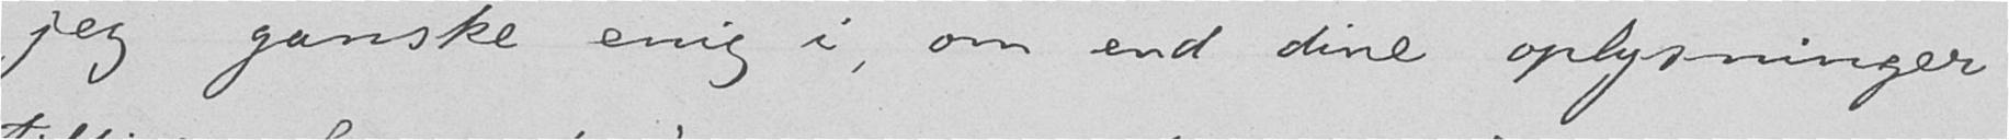jeg ganske enig i, om end dine oplysninger
